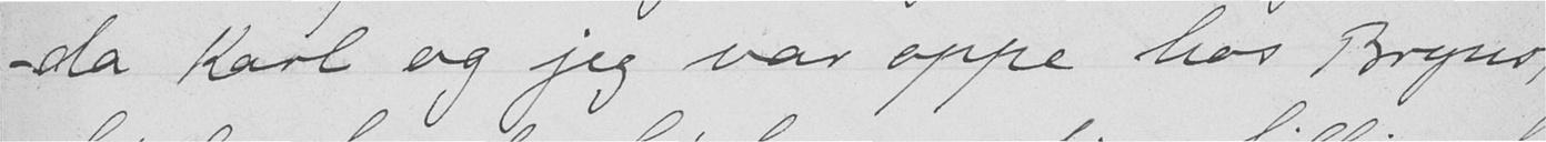da Karl og jeg var oppe hos Bryns,
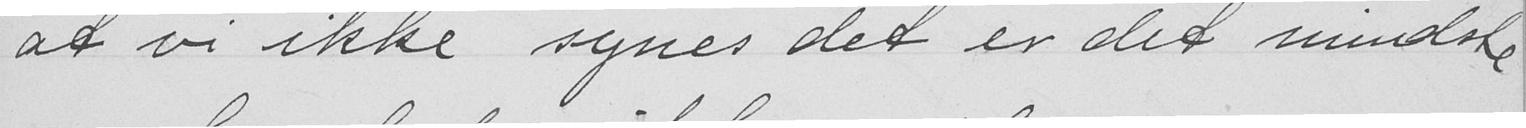at vi ikke synes det er det mindste
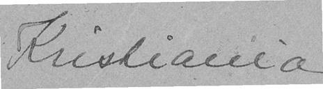Kristiania
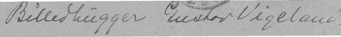Billedhugger Gustav Vigeland
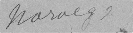Norvege
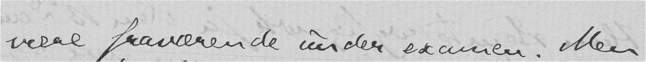være fraværende under examen. Men
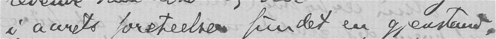i aarets foreteelser fundet en gjenstand,
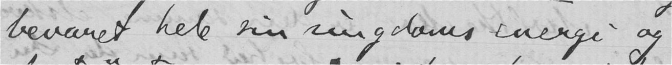bevaret hele sin ungdoms energi og
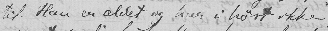til. Han er ældet og har i høst ikke
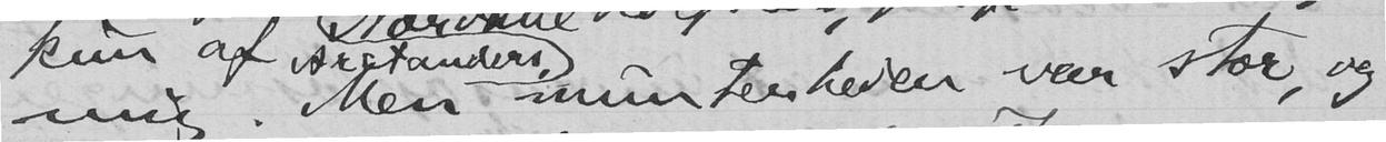mig. Men munterheden var stor, og
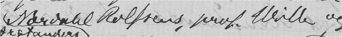Nordahl Rolfsens, prof. Wille og
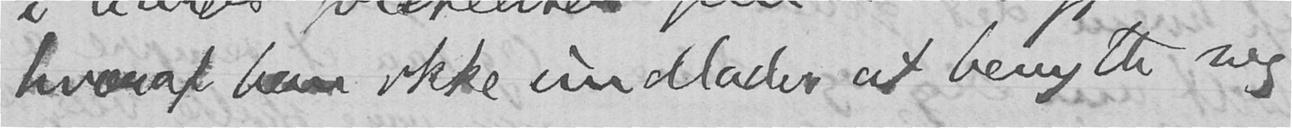hvoraf han ikke undlader at benytte sig.
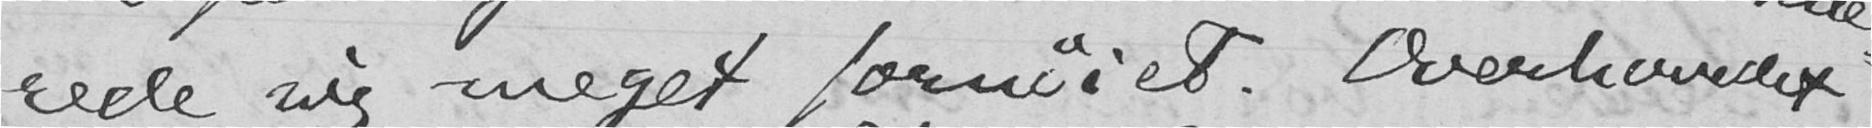rede sig meget fornøiet. Overhovedet
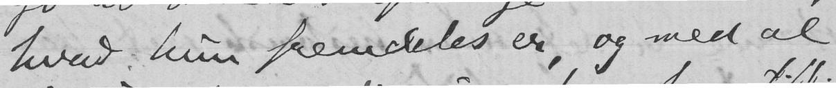hvad hun fremdeles er, og med al
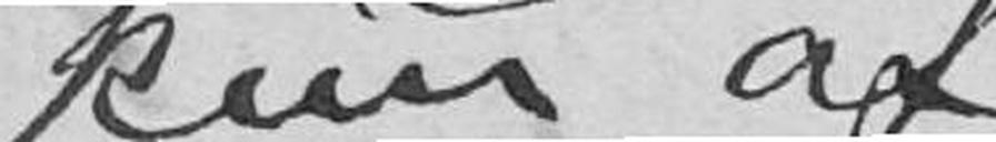kun af
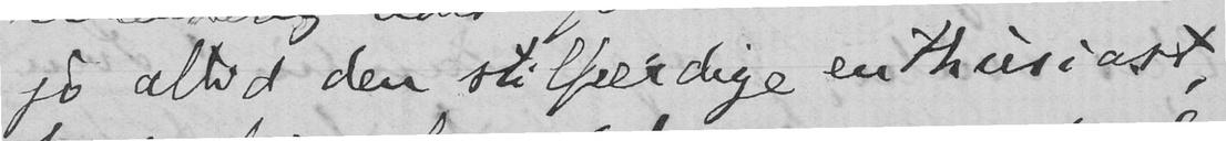jo altid den stilfærdige enthusiast,
